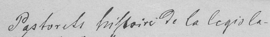Pastorcks Histoire de la Legisla-
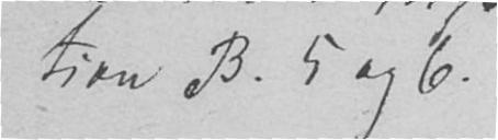tion B. 5 og 6.
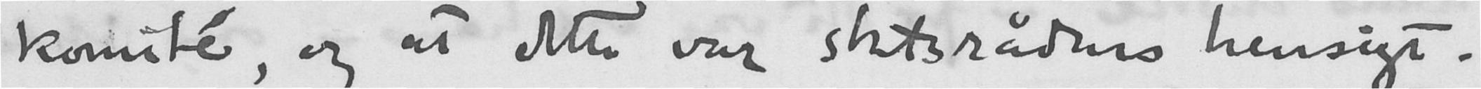komité, og at dette var statsrådens hensigt.
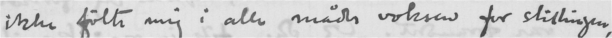ikke følte mig i alle måder voksen for stillingen,
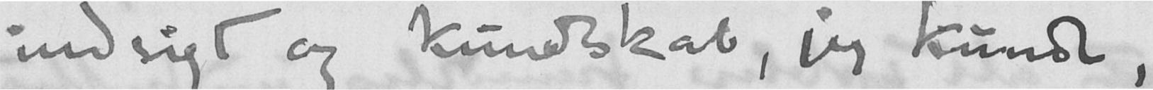indsigt og kundskab, jeg kunde,
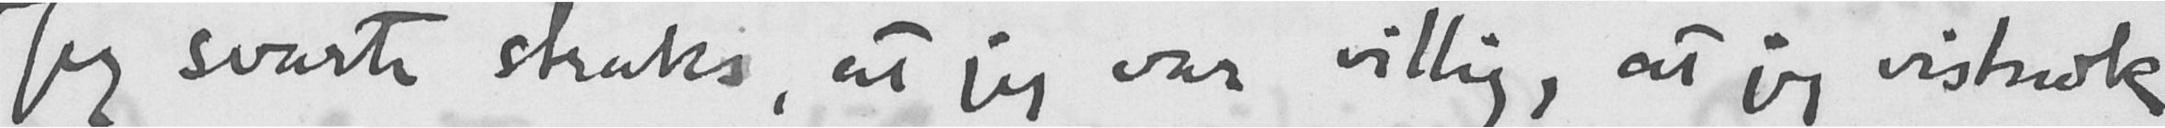Jeg svarte straks, at jeg var villig, og at jeg vistnok
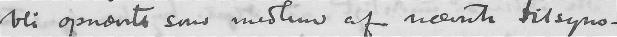bli opnævnt som medlem af nævnte tilsyns-
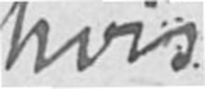hvis
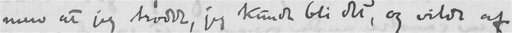men at jeg trodde, jeg kunde bli det, og vilde af
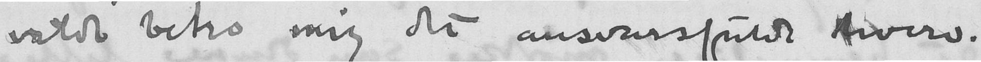vilde betro mig det ansvarsfulde hverv.
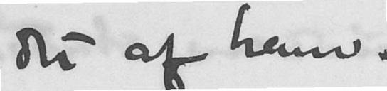det af ham.
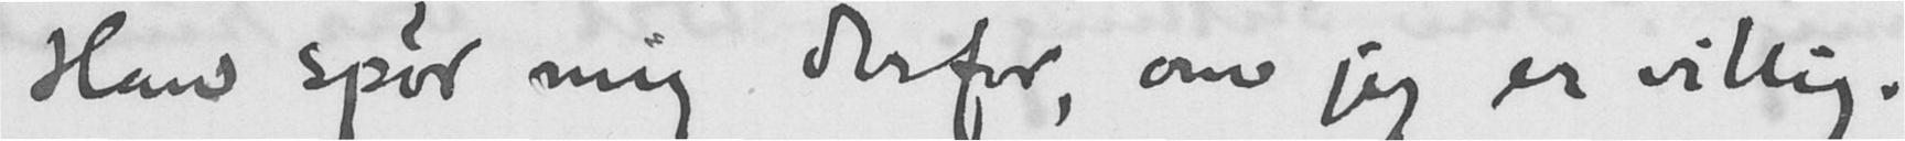Han spør mig derfor, om jeg er villig.
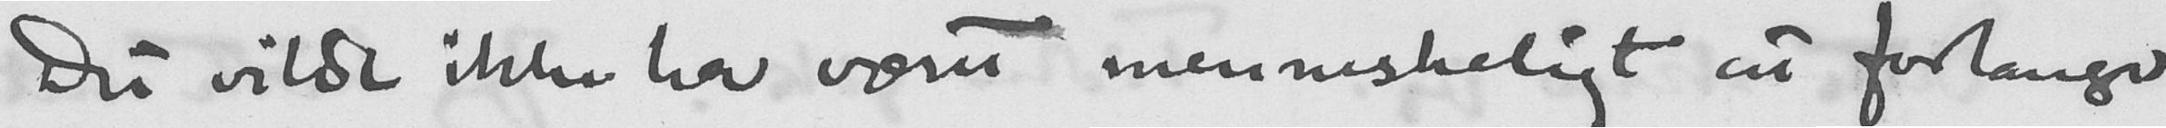Det vilde ikke ha været menneskeligt at forlange
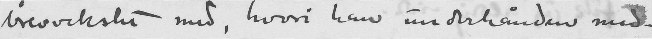brevvekslet med, hvori han underhånden med-
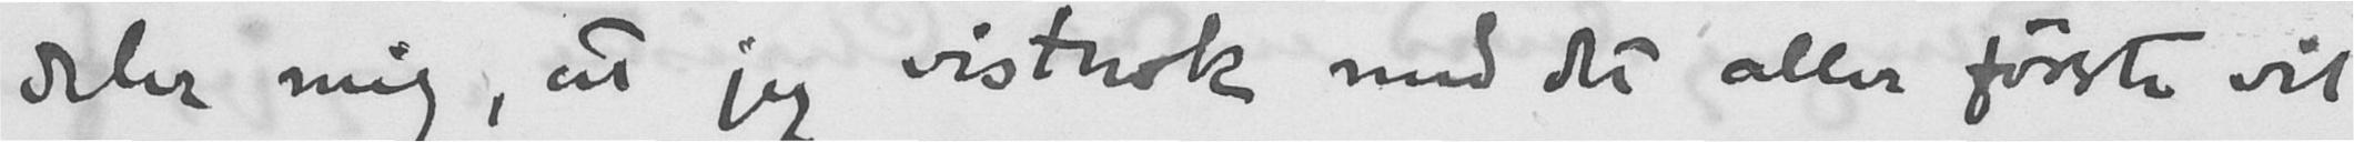deler mig, at jeg vistnok med det aller første vil
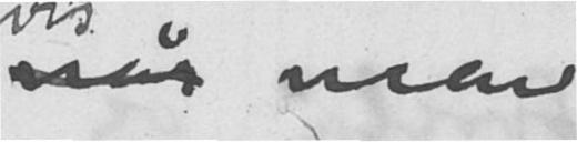når man
